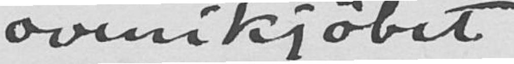ovenikjøbet
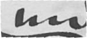ind
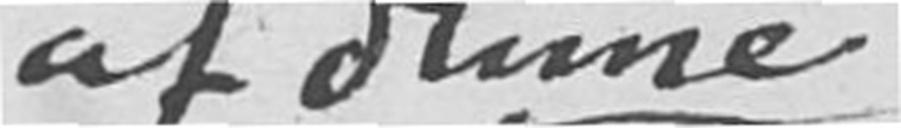af denne
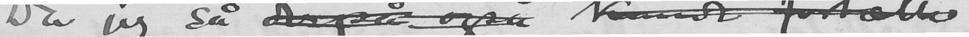Da jeg så derpå også kunde fortælle
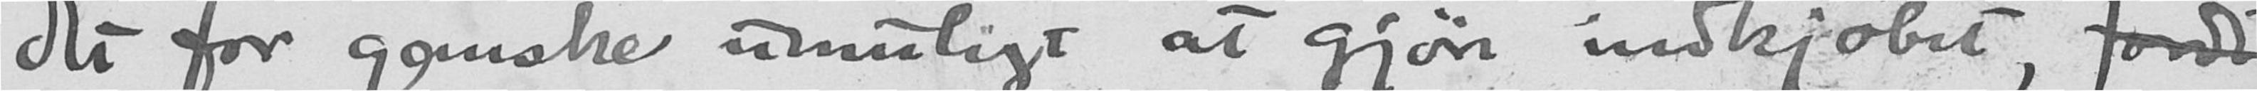det for ganske umuligt at gjøre indkjøbet, fordi
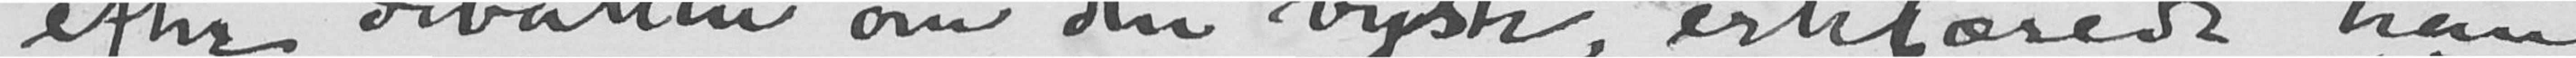efter debatten om din byste, erklærede han
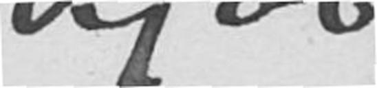kjøb
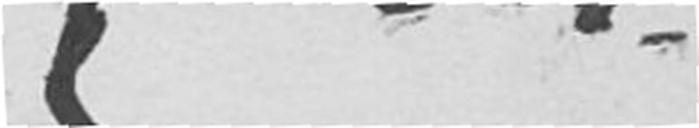(hvor-
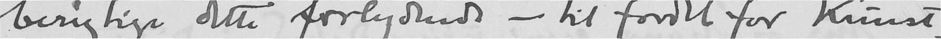berigtige dette forlydende - til fordel for Kunst-
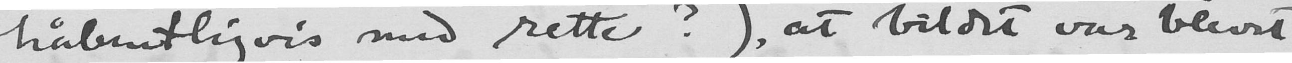håbentligvis med rette?) at bildet var blevet
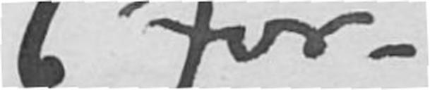(for-
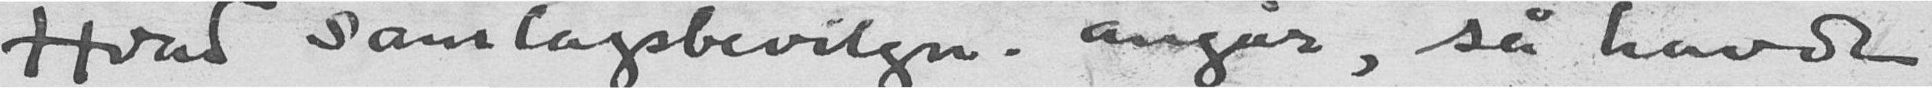Hvad samlagsbevilgn. angår, så havde
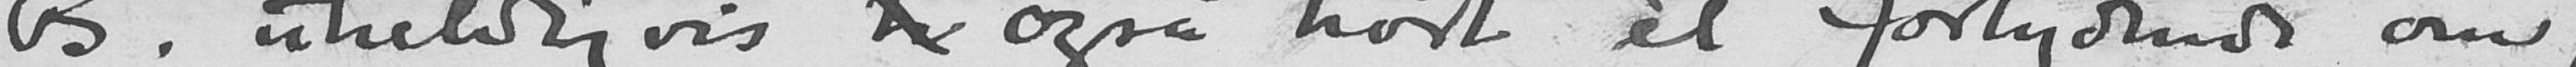B. uheldigvis h også hørt et forlydende om,
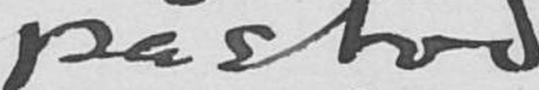påstod
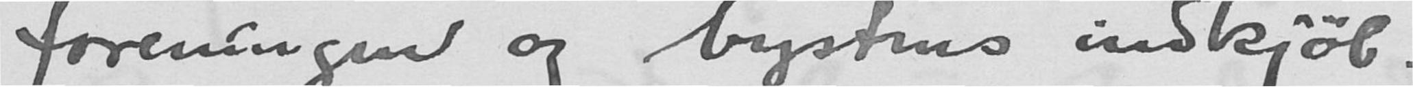foreningen og bystens indkjøb.
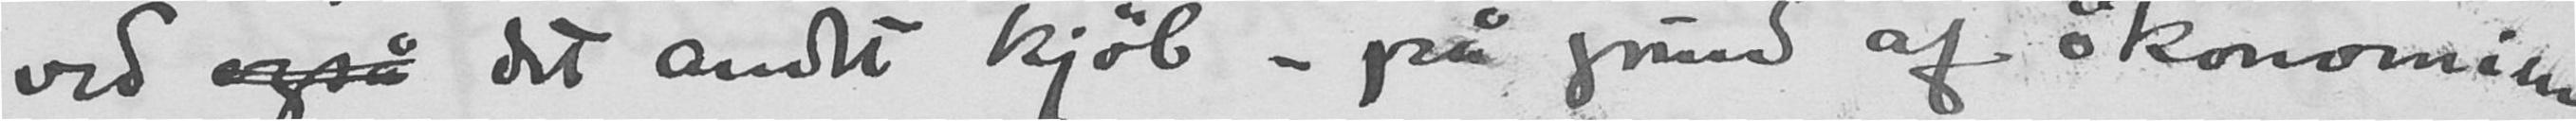ved også det andet kjøb - på grund af økonomien
